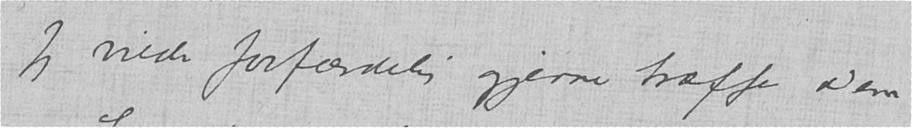Jeg vilde forfærdelig gjerne træffe Dem
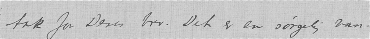tak for Deres brev. Det er en sørgelig van-
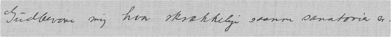Gudbevare mig hvor skrækkelige saanne sanatorier er.
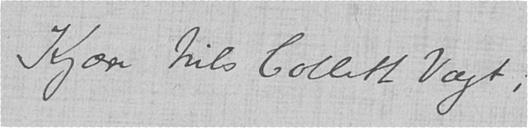Kjære Nils Collett Vogt,
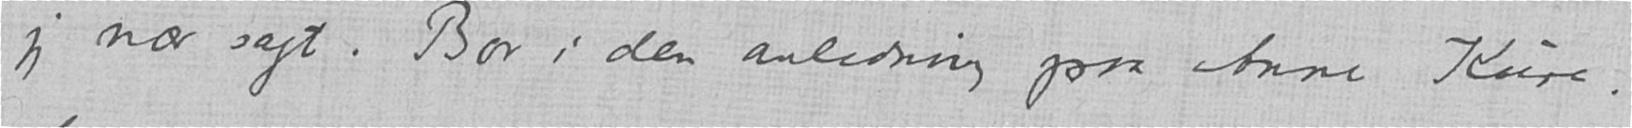jeg nær sagt. Bor i den anledning paa Anne Kure.
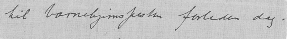til barnehjemsfesten forleden dag.
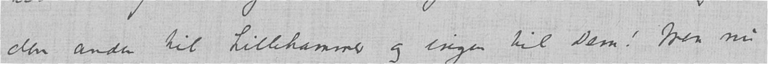den anden til Lillehammer og ingen til Dem! Men nu
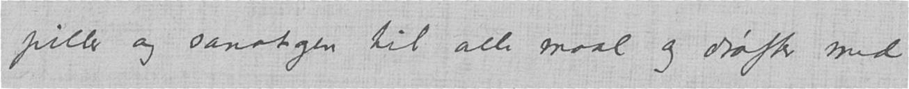piller og sanatogen til alle maal og drøfter med
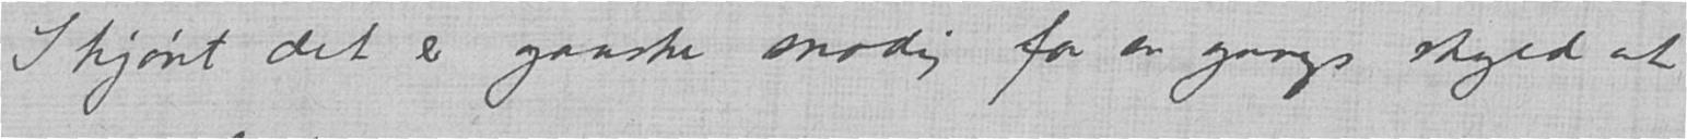Skjønt det er ganske snodig for en gangs skyld at
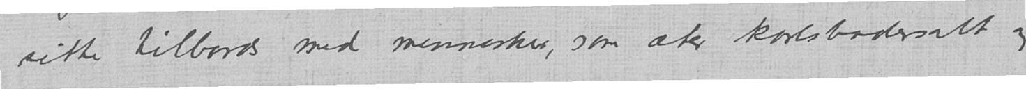sitte tilbords med mennesker, som æter karlsbadersalt og
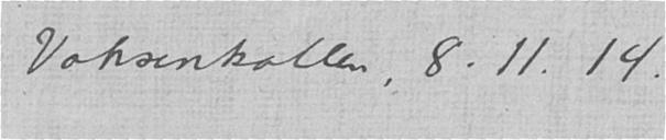Voksenkollen, 8.11.14.
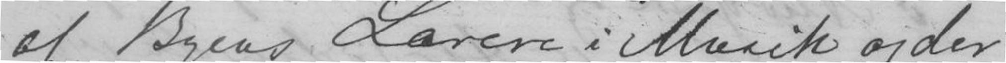af Byens Lærere i Musik og der
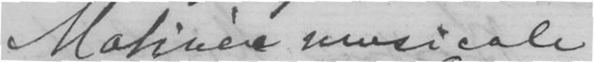Matinée musicale af two a three
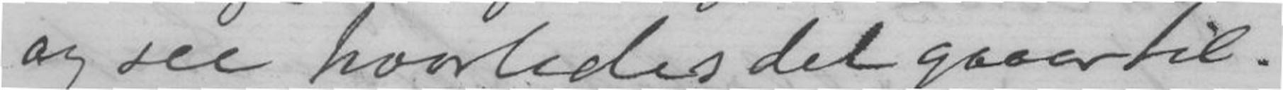og see hvorledes det gaaer til.
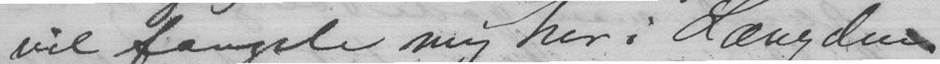vil fængsle mig her i Længden.
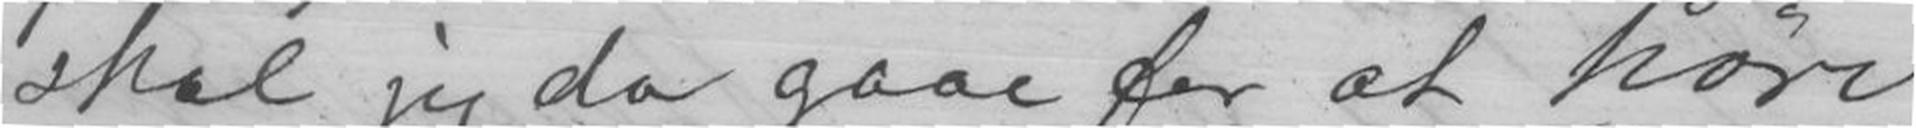skal jeg da gaae for at høre
